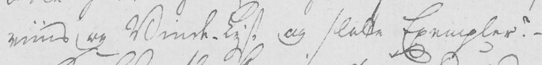viins og Vinde-Lyst og slette Exempler. -
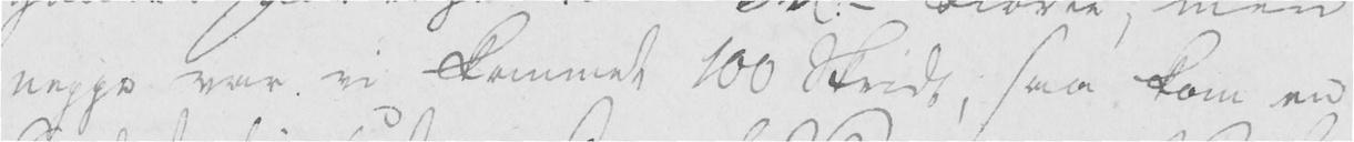neppe var vi kommet 100 Skridt, saa kom en
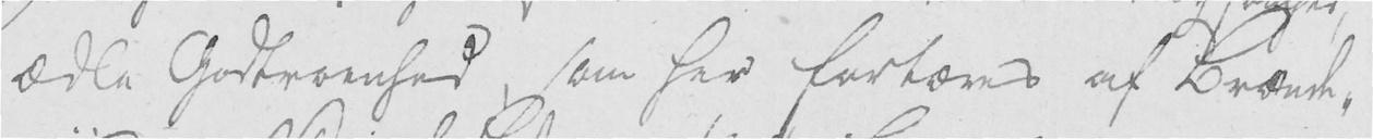ædle Godtroenhed, som her fortæres af Brænde-
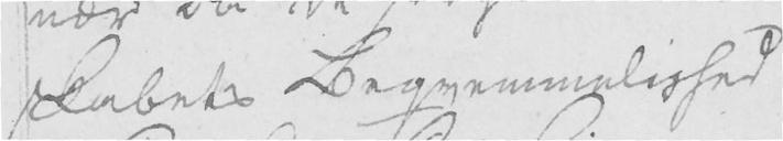skabets Beqvemmelighed
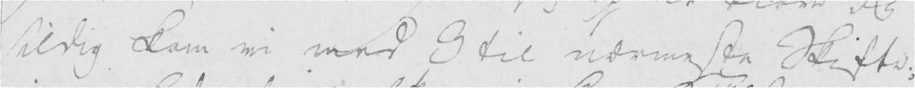sildig kom vi med 3 til nærmeste Skifte;
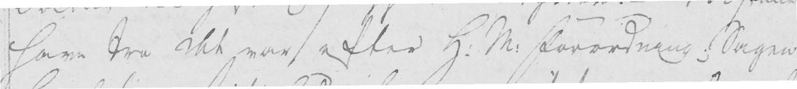have tre det var, efter H. M. Forordning; Sagen
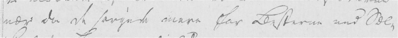nær da de sørgede mere for Hesterne end Sæl-
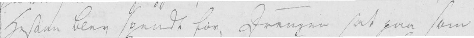Hesten blev spendt for, Drengen sat paa som
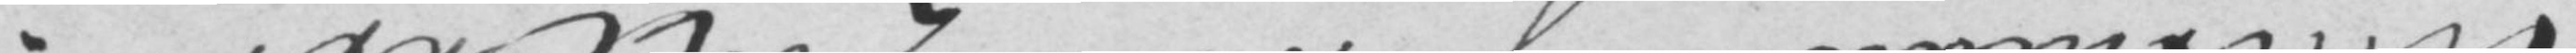Amtmand Johan Collett.
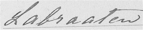Labraaten
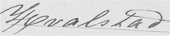Hvalstad
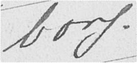borg.
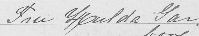Fru Hulda Gar-
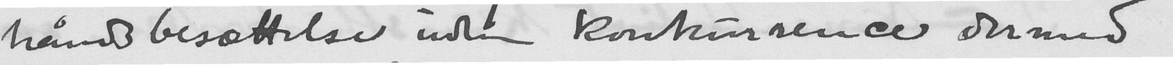håndsbesættelse uden konkurrence dermed
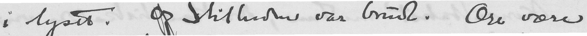i lyset. Og Stilheden var brudt. Ære være
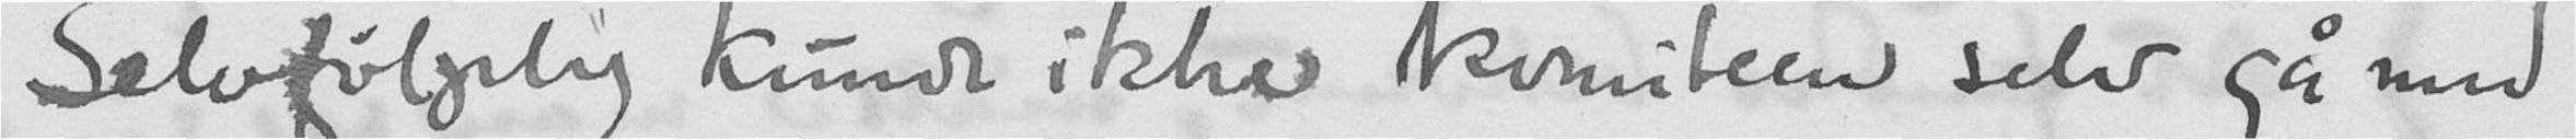Selvfølgelig kunde ikke komiteen selv gå med
